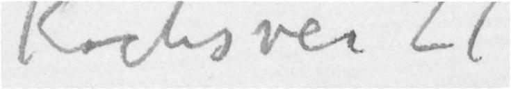Kochsvei 21
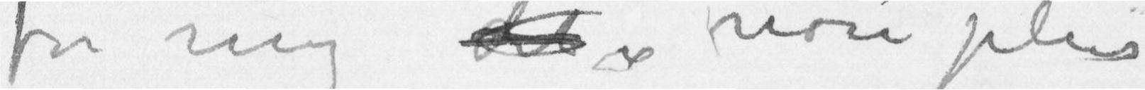for mig det non plus
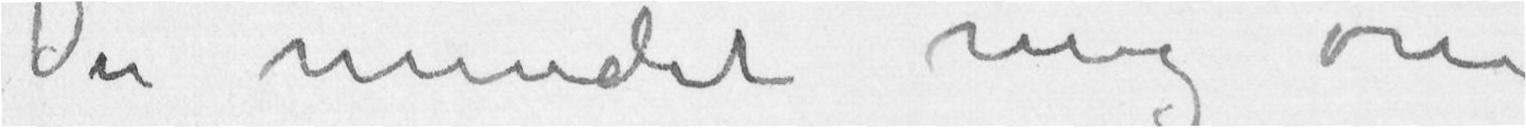Du mindet mig om
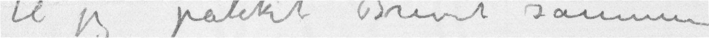til jeg pakket Brevet sammen
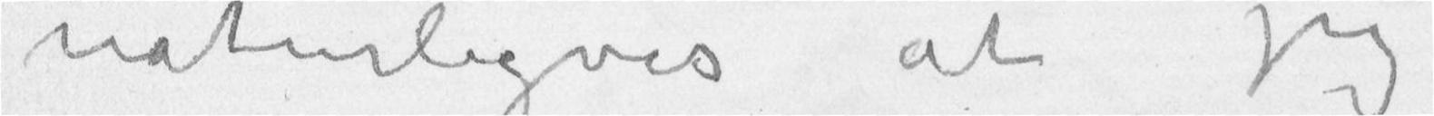naturligvis at jeg
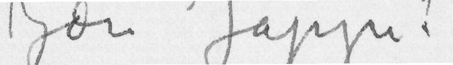Kjære Jappe!
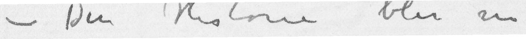– Din Historie blir nu
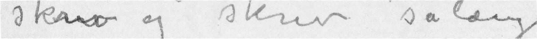skrev og skrev sålænge
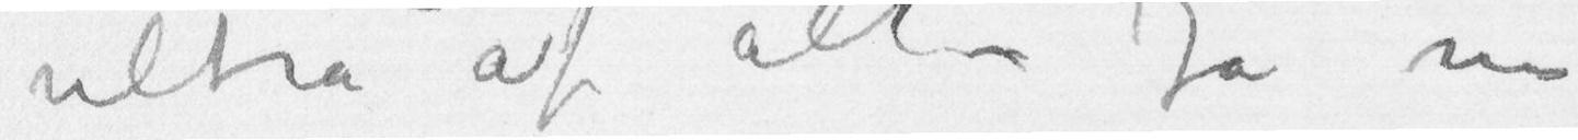ultra af alt – Ja nu
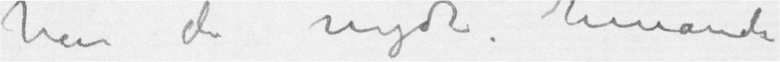kan de nyde hinanden
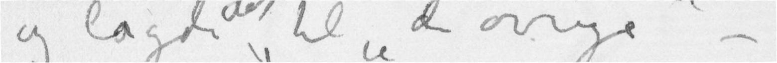og lagde det til «de øvrige» –
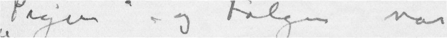«Pigen» og Følgen var
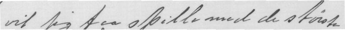vil jeg faa spille med de største
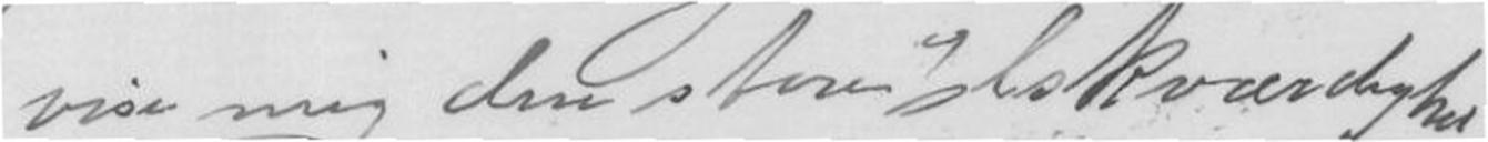vise mig den store ælskværdighed
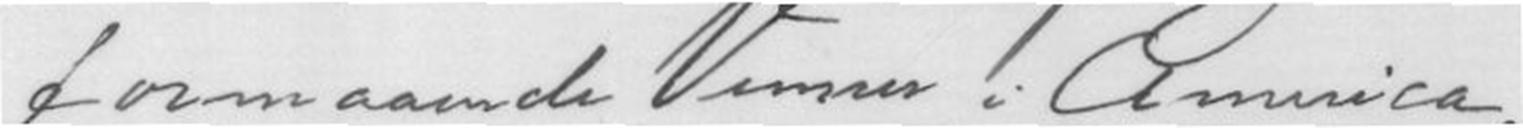formaaende Venner i America
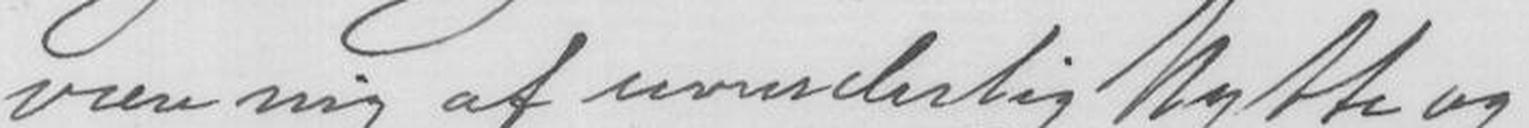være mig af uvurderlig Nytte og
